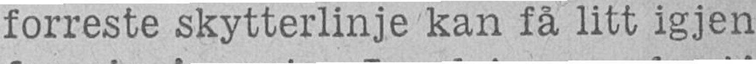forreste skytterlinje kan få litt igjen
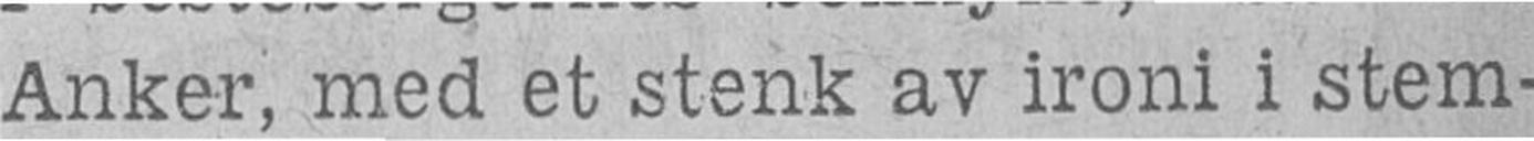Anker, med et stenk av ironi i stem-
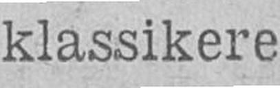klassikere - - -
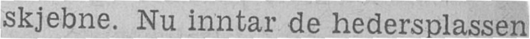skjebne. Nu inntar de hedersplassen
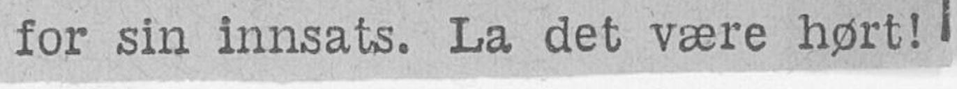for sin innsats. La det være hørt!
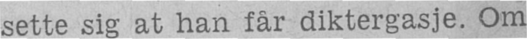sette sig at han får diktergasje. Om
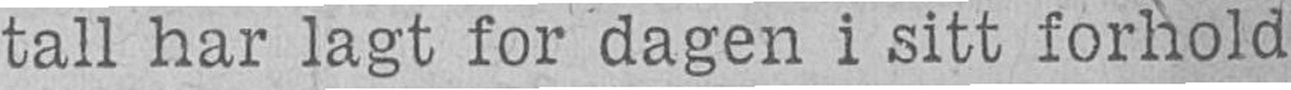tall har lagt for dagen i sitt forhold
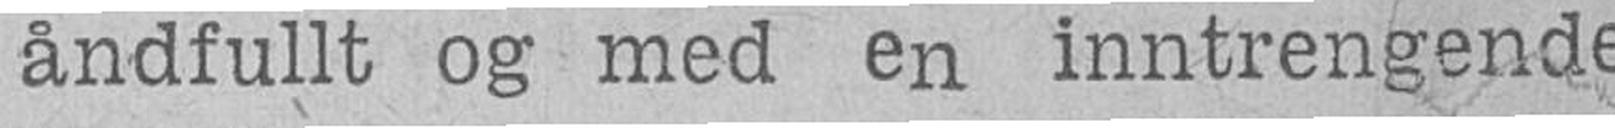åndfullt og med en inntrengende
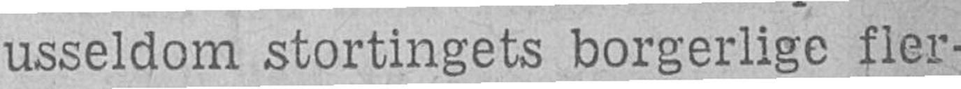usseldom stortingets borgerlige fler-
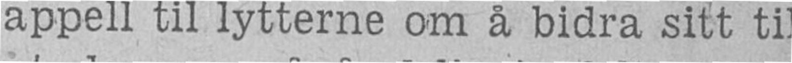appell til lytterne om å bidra sitt til
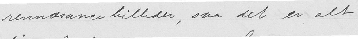rennæsancebilleder, saa det er alt
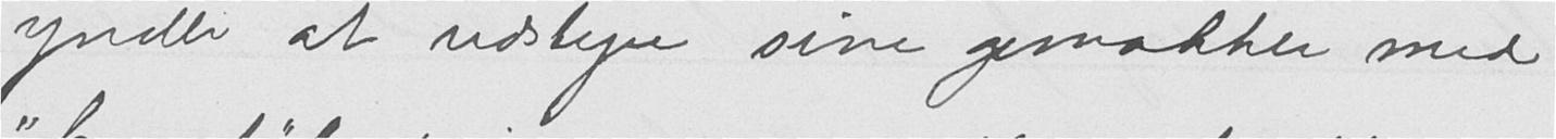ynder at udstyre sine gemakker med -
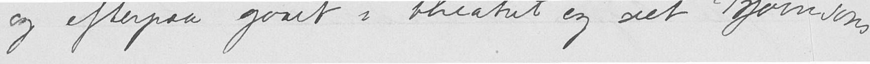og efterpaa gaaet i theatret og seet Bjørnsons
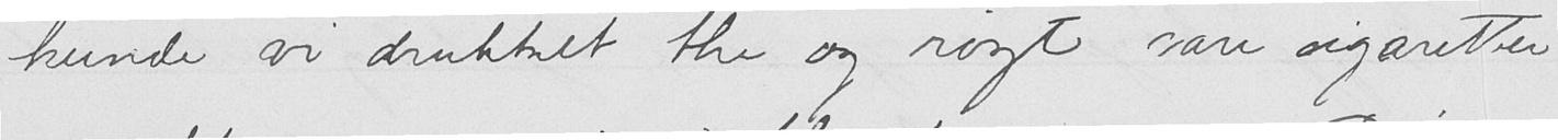kunde vi drukket the og røgt vore cigaretter
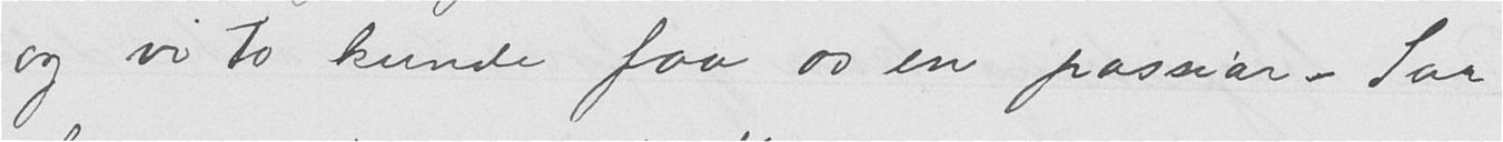og vi to kunde faa os en passiar - Saa
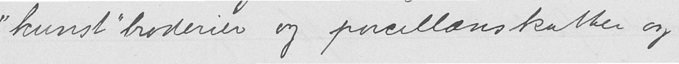"kunst" broderier og porcellænskatter og
